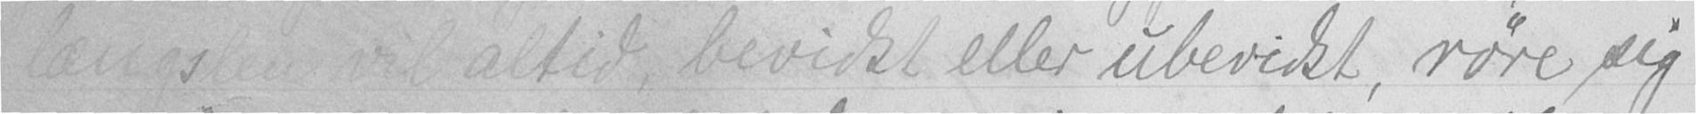længslen vil altid, bevidst eller ubevidst, røre sig
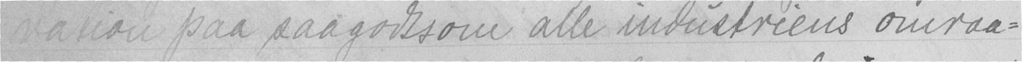vation paa saagodtsom alle industriens omraa-
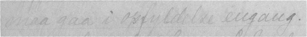maa gaa i opfyldelse engang.
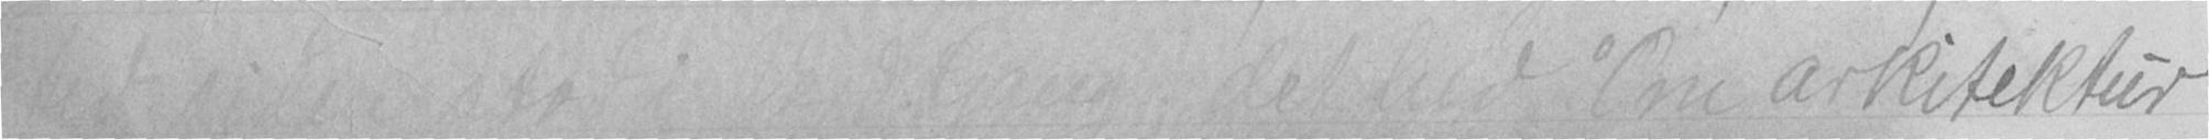stund siden sto i Verdens Gang; det hed "Om arkitektur
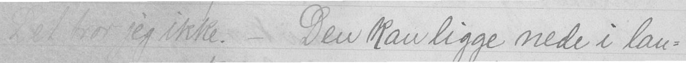Det tor jeg ikke. - Den kan ligge nede i lan-
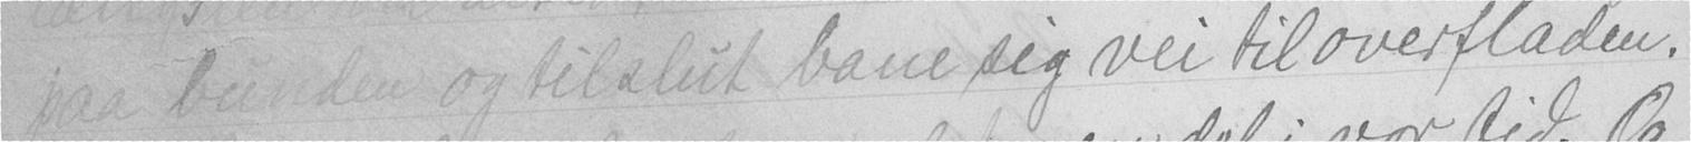paa bunden og tilslut bane sig vei til overfladen.
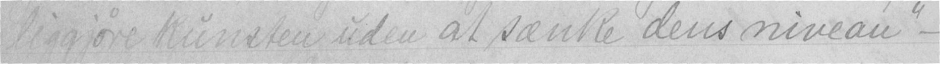liggjøre kunsten uden at sænke dens niveau" -
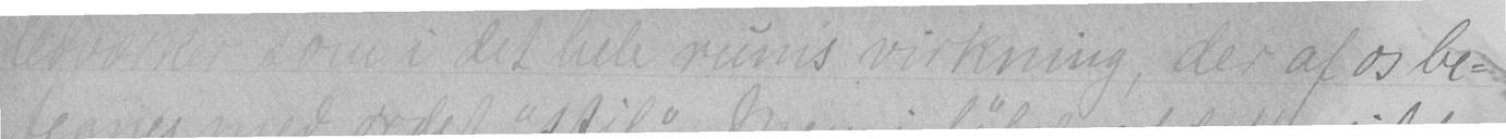bedværker som i det hele rums virkning, der af os be-
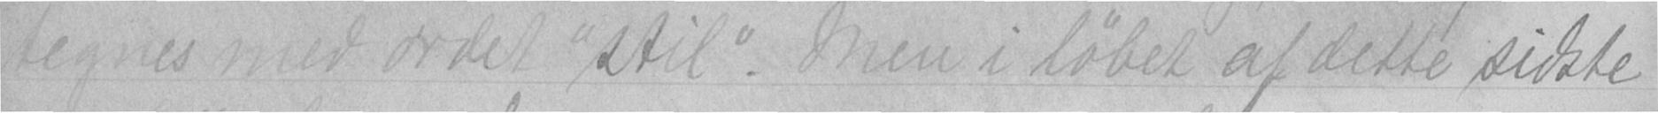tegnes med ordet "stil". Men i løbet af dette sidste
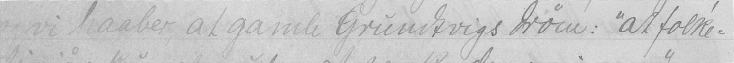og vi haaber, at gamle Grundtvigs drøm: "at folke-
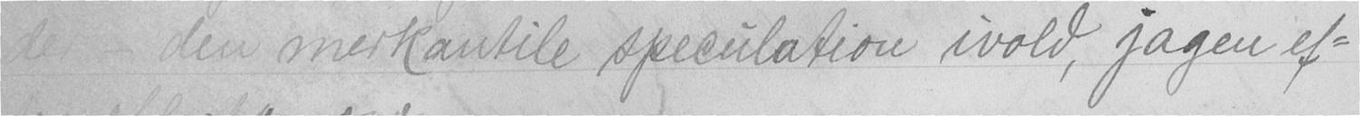der - den merkantile speculation ivold, jagen ef-
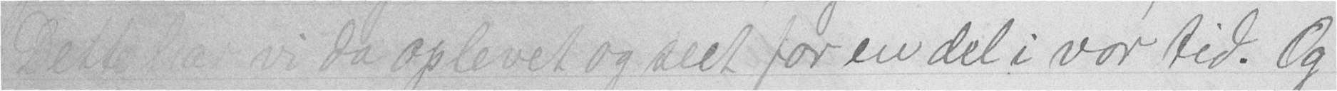Dette her vi da oplevet og seet for en del i vor tid. Og
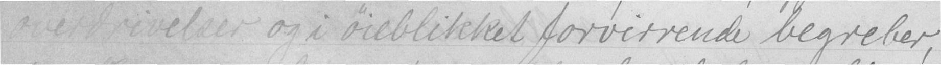overdrivelser og i øieblikket forvirrende begreber,
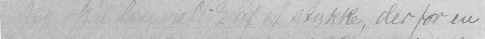Jeg skal læse op lidt af et stykke, der for en
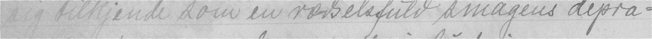sig tilkjende som en rædselsfuld smagens depra-
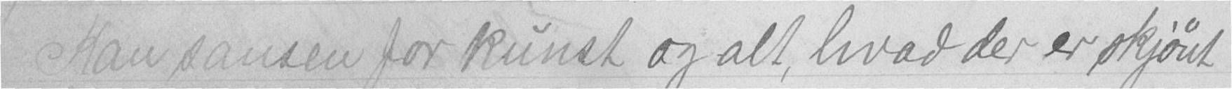Kan sansen for kunst og alt, hvad der er skjønt
